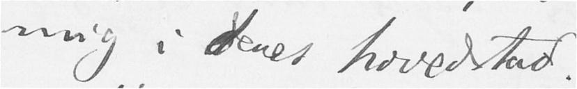mig i deres hovedstad.
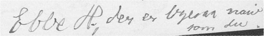Ebbe H., der er ligesaa naiv
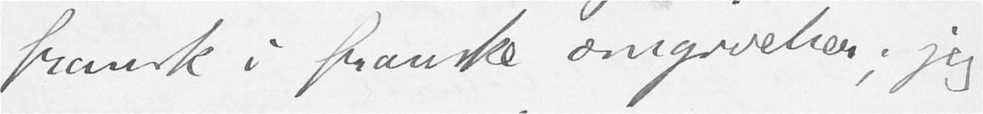fransk i franske omgivelser; jeg
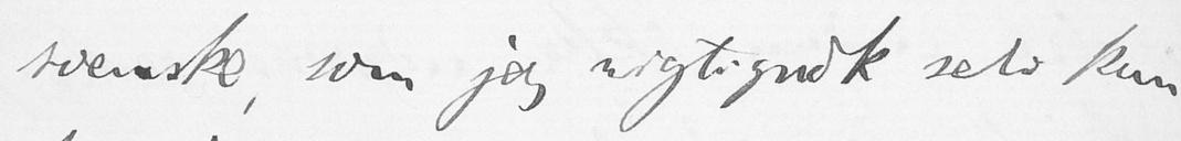svenske, som jeg rigtignok selv kun
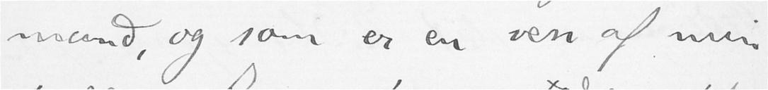mand, og som er en ven af min
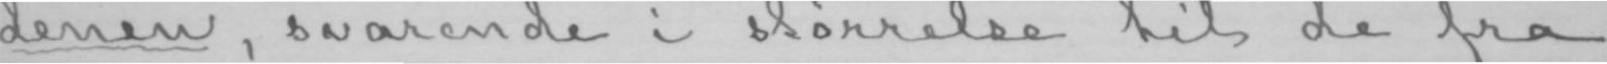denen, svarende i størrelse til de fra
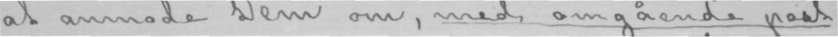at anmode Dem om, med omgående post
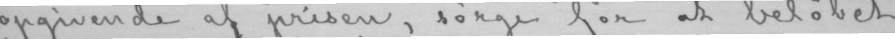opgivende af prisen, sørge for at beløbet
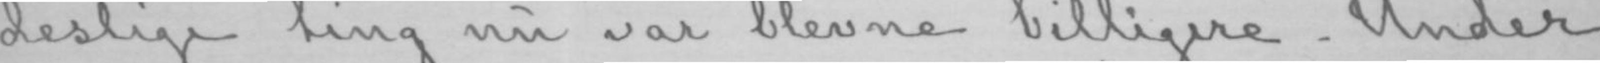deslige ting nu var blevne billigere. Under
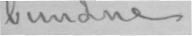bundne
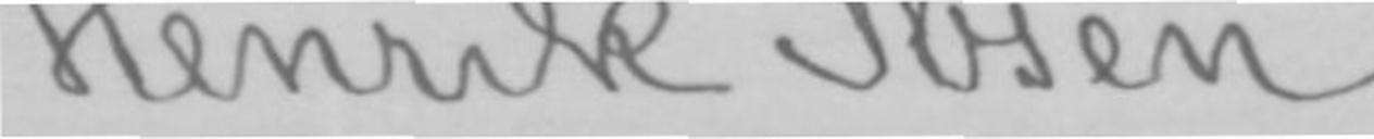Henrik Ibsen.
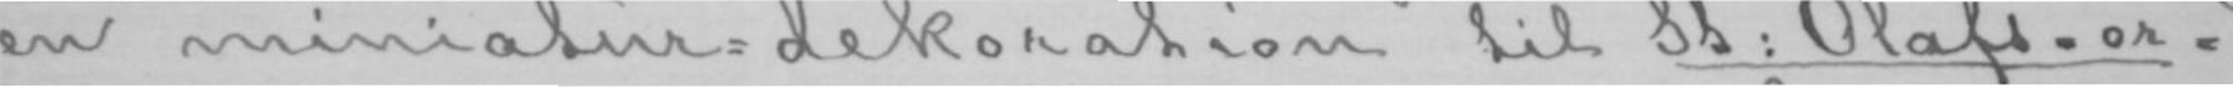en miniatur-dekoration til St: Olafs-or-
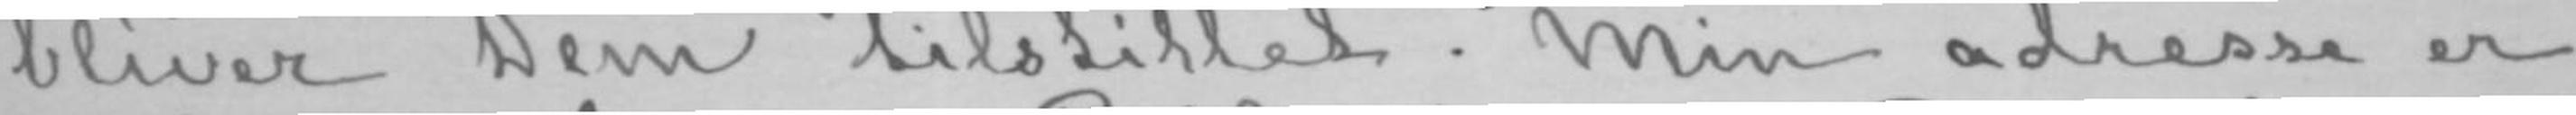bliver Dem tilstillet. Min adresse er
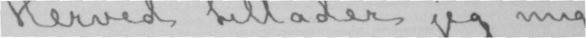Herved tillader jeg mig
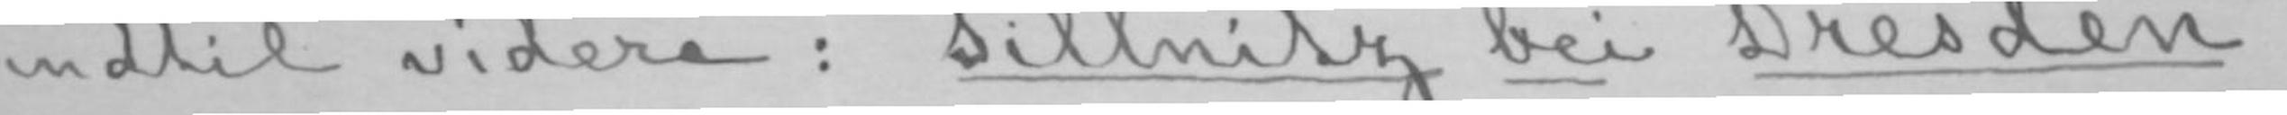indtil videre: Pillnitz bei Dresden.
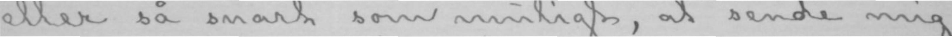eller så snart som muligt, at sende mig
